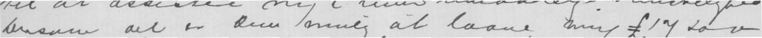Dersom det er Dem mulig at laane mig £17 saa
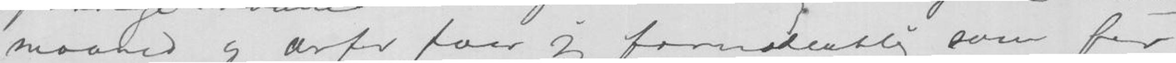maaned og derfor faar jeg formodentlig som før
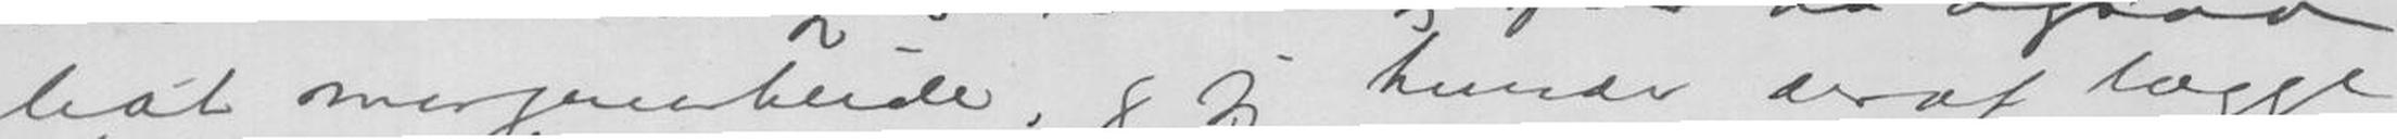lidt morgenarbeide, og jeg kunde der af lægge
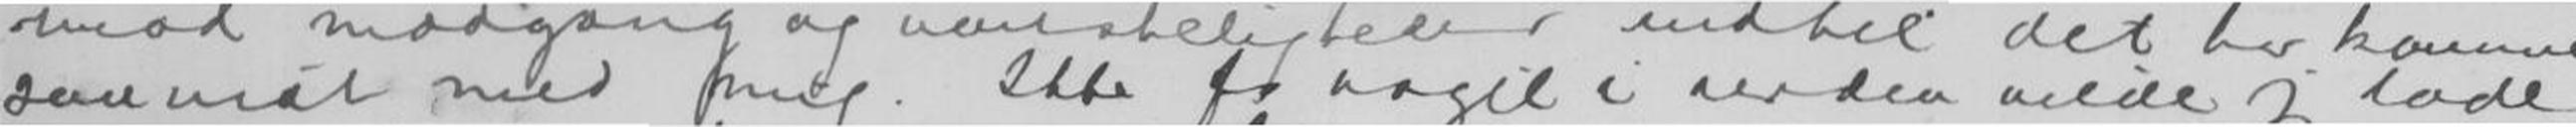saa vidt med mig. Ikke for noget i verden vilde jeg lade
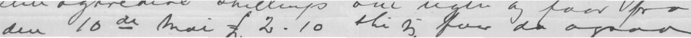den 10de Mai £2.10 thi jeg faar da ogsaa
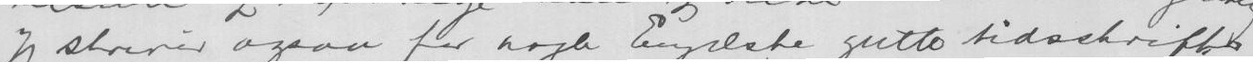Jeg skriver ogsaa for nogle Engelske gutte tidskrifter
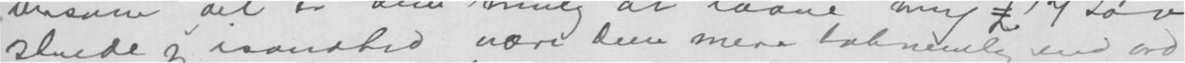skulde jeg isandhed være Dem mere taknemlig end ord
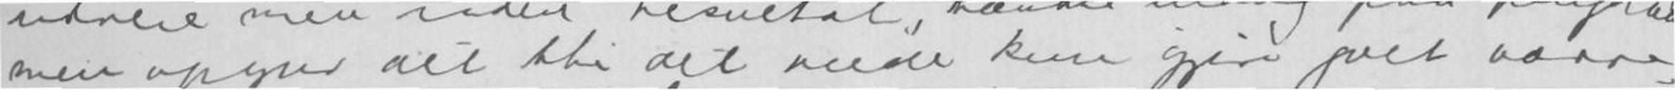men opgav det thi det vilde kun gjøre galt værre
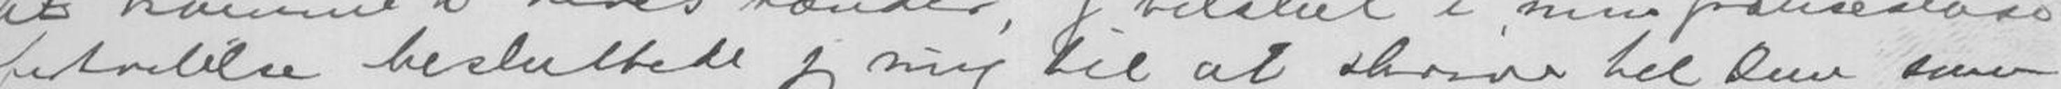fortvilelse besluttede jeg til at skrive til Dem som
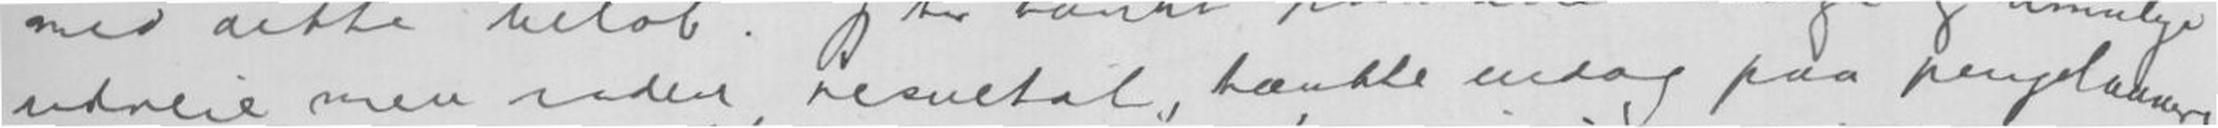udveie men uden resultat, tænkte endog paa pengelaanere
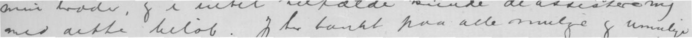med dette beløb. Jeg har tænkt paa alle mulige og umulige
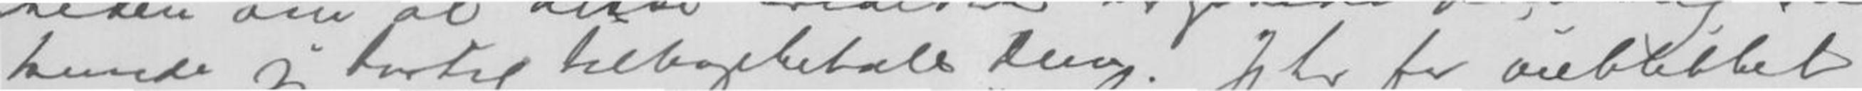kunde jeg hurtig tilbagebetale Dem. Jeg har for øieblikket
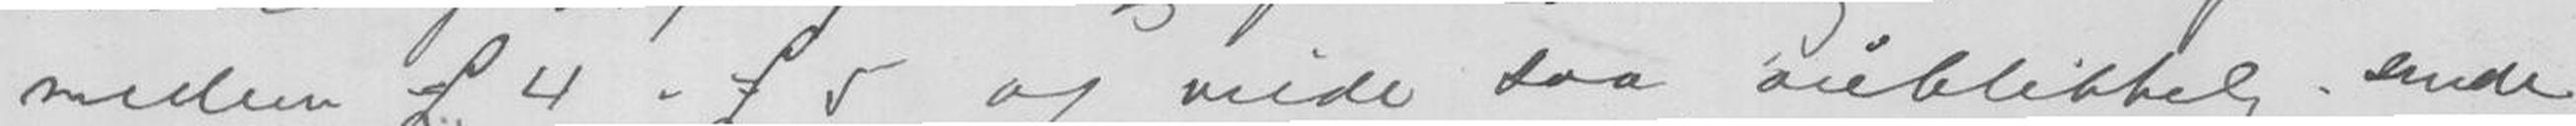medem £4-£5 og vilde saa øieblikkelig sende
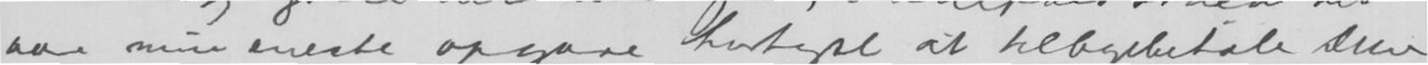være min eneste opgave hurtigst at tilbagebetale Dem
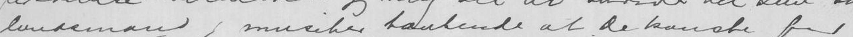landsmand, musiker tænkende at De kanske for
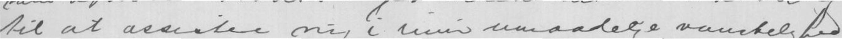til at assistere mig i min umaadelige vanskelighed.
